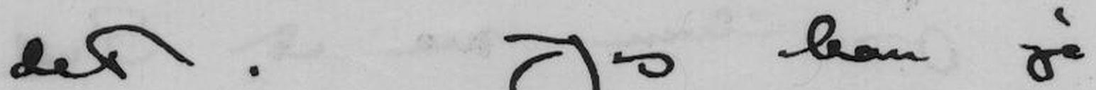det. Jeg kan jo
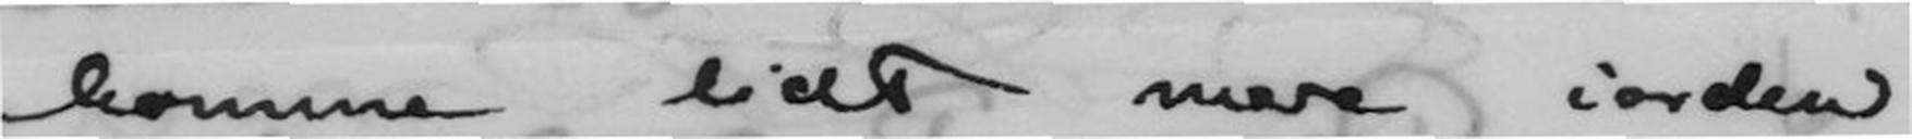komme lidt mere iorden
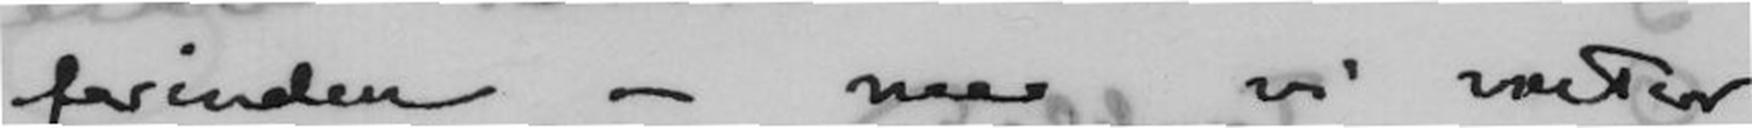forinden - naar vi venter.
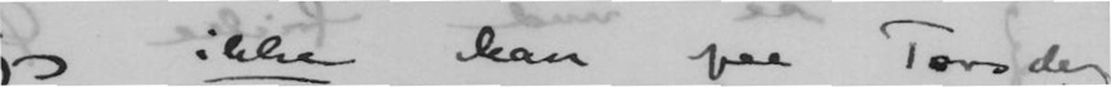jeg ikke kan paa Torsdag
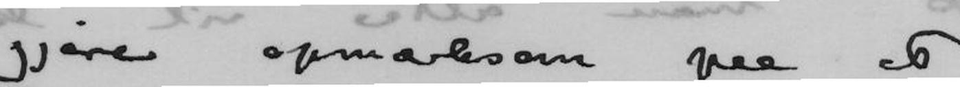gjøre opmærksom paa at
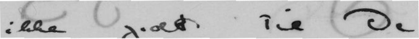ikke godt til De
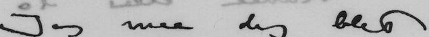Jeg maa dog blot
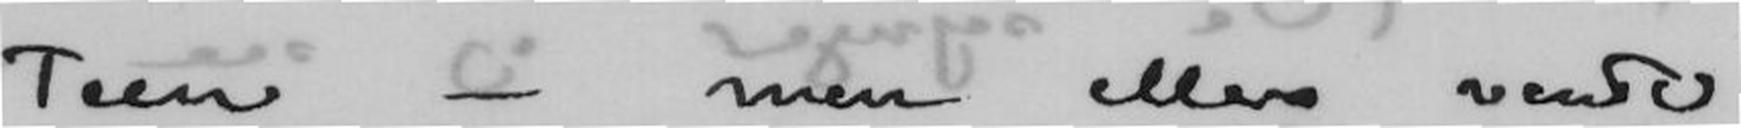teen - men ellers vendter
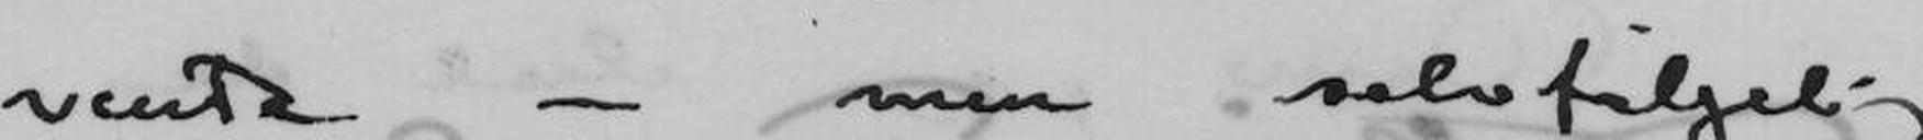vente - men selvfølgelig
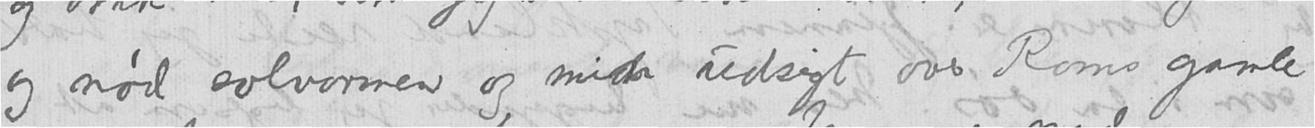og nød solvarmen og min udsigt over Romas gamle
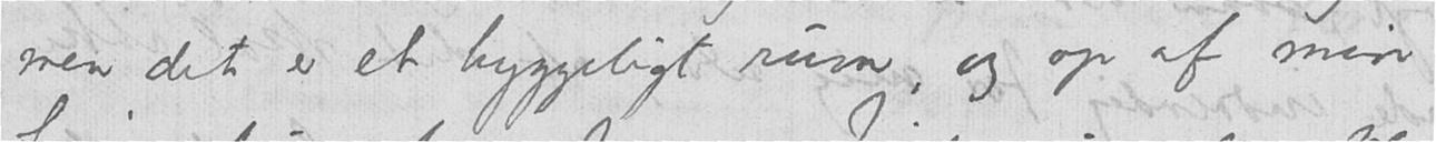men det er et hyggeligt rum, og op af
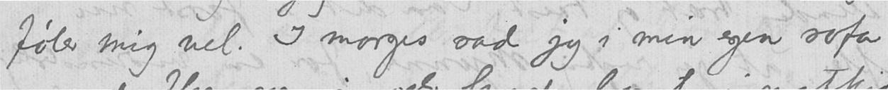føler mig vel. I morges sad jeg i min egen sofa
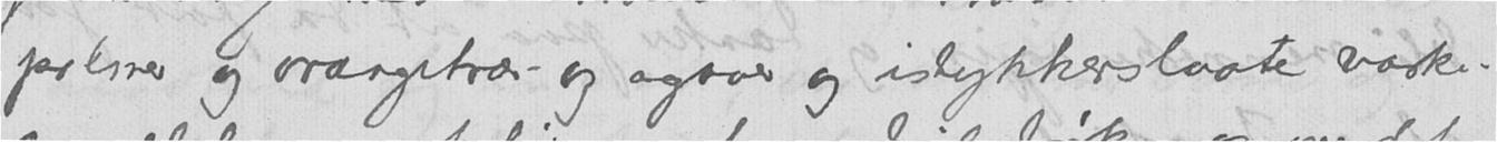palmer og orangetrær - og agaver og istykkerslaate vaske-
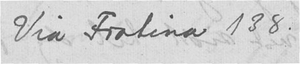Via Fratina 138.
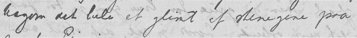bakom det hele et glimt af stenegene paa
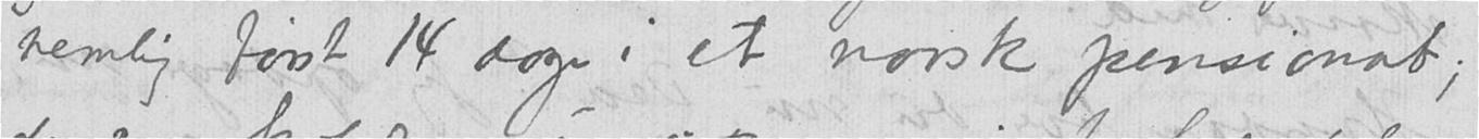nemlig først 14 dage i et norsk pensionat;
